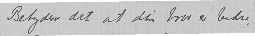Betyder det at din bror er bedre,
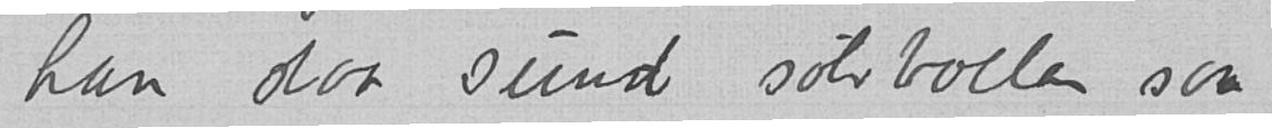han slaa sund sølvbollen saa
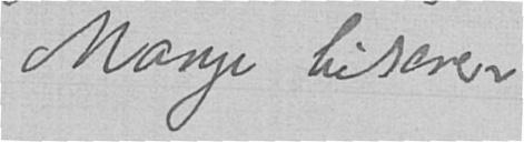Mange hilsener
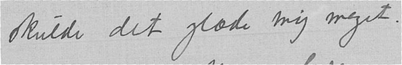skulde det glæde mig meget.
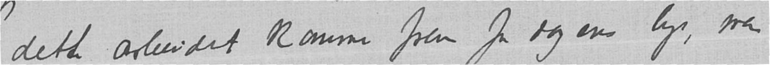dette arbeidet komme frem for dagens lys, men
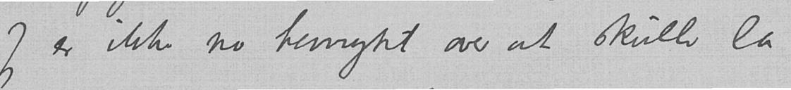Jeg er ikke no henrykt over at skulle la
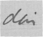din
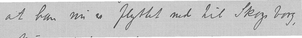at han nu er flyttet ned til Skogsborg,
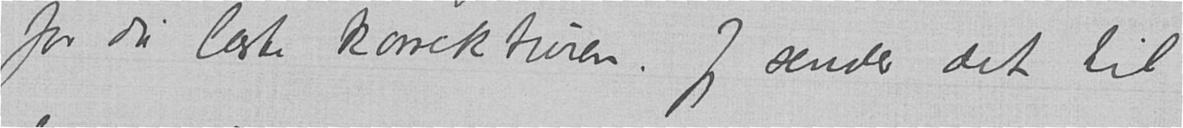for du læste korrekturen. Jeg sender det til
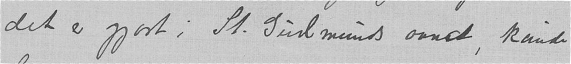det er gjort i St. Gudmunds aand, kunde
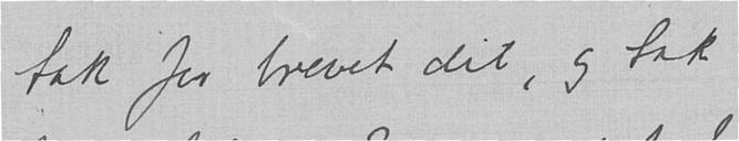tak for brevet dit, og tak
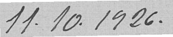11.10.1926
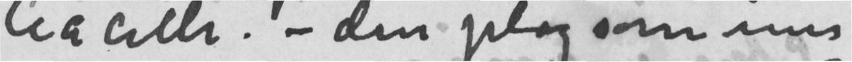Ciacelli. – den plagsomme
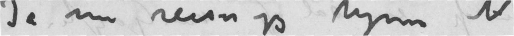Ja nu reiser jeg hjem til
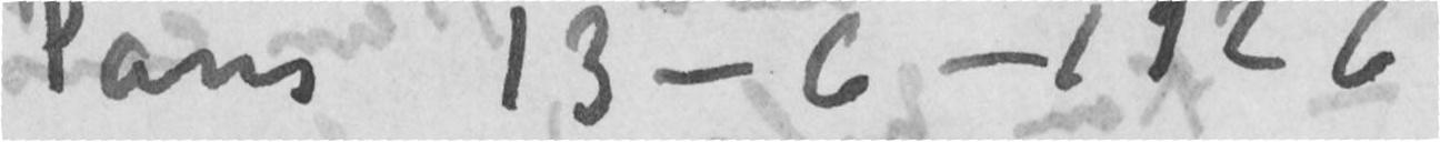Paris 13–6–1926
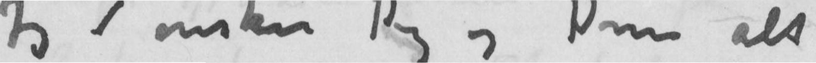Jegønsker Dig og Dine alt
